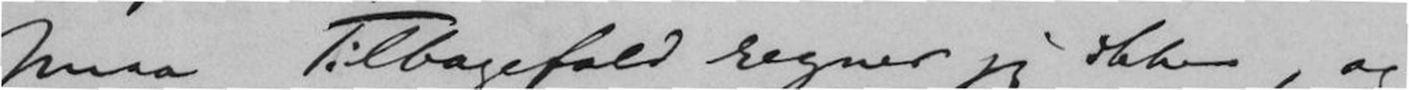smaa Tilbagefald regner jeg ikke, og
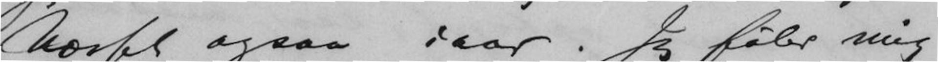Næsset ogsaa iaar. Jeg føler mig
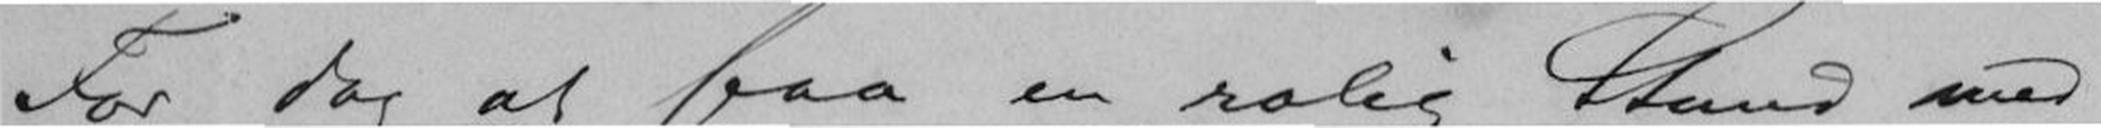For dog at faa en rolig Stund med
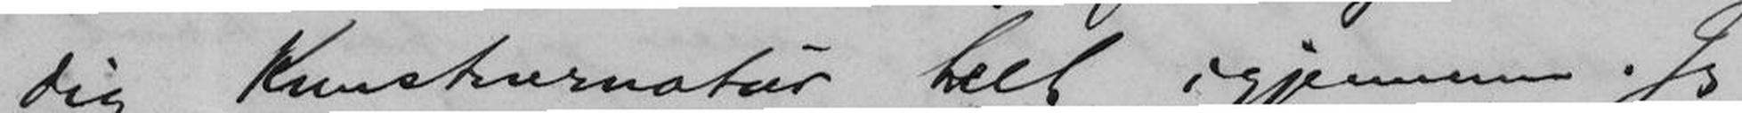dig Kunstnernatur helt igjennem. Jeg
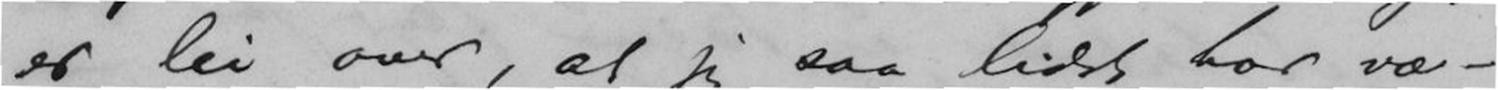er lei over, at jeg saa lidet har væ-
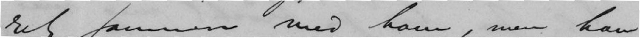ret sammen med ham, men
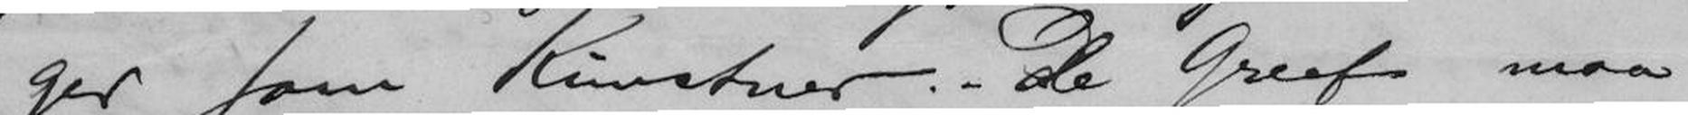ger som Kunstner. De Greef maa
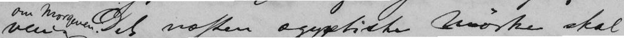Det næsten ægyptiske Mørke skal
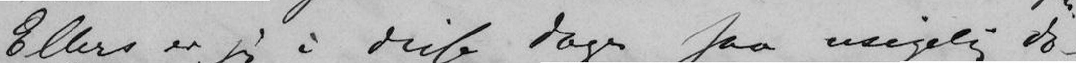Ellers er jeg i disse Dage saa usigelig do-
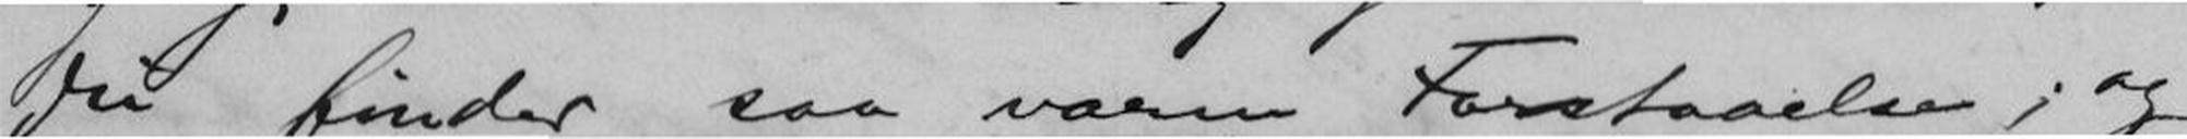du finder saa varm Forstaaelse; og
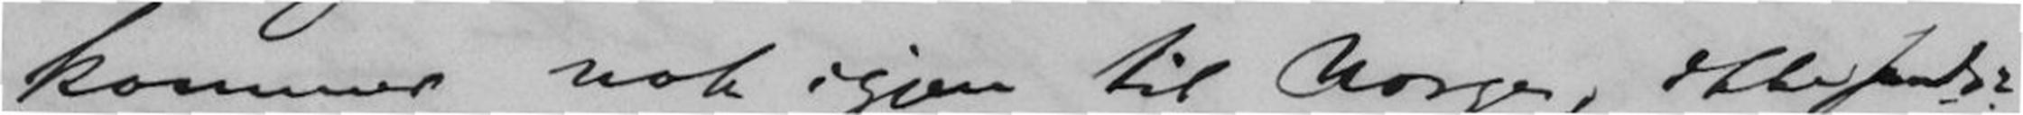han kommer nok igjen til Norge, ikkesandt?
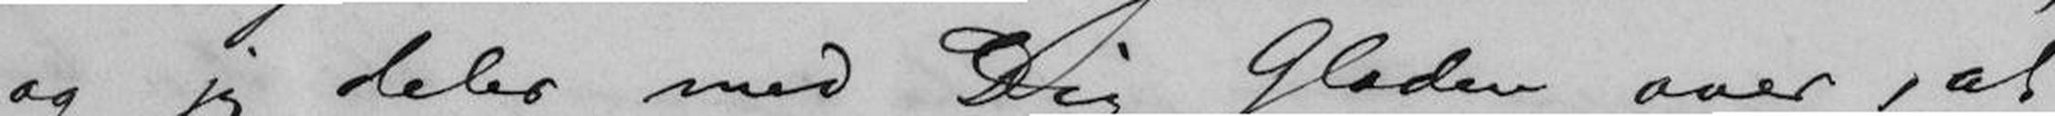og jeg deler med Dig Glæden over, at
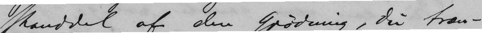standdel af den Gjødning, du træn-
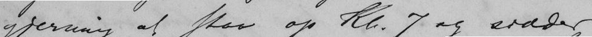gjerning at staa op Kl. 7 og sidder
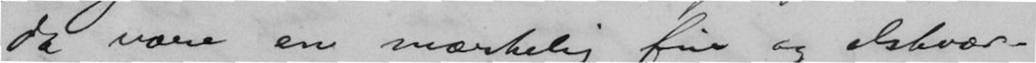da være en mærkelig fin og elskvær-
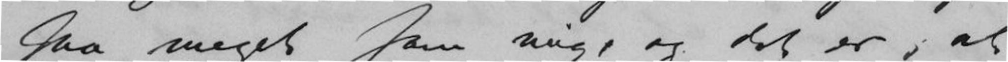meget som mig, og det er, at
